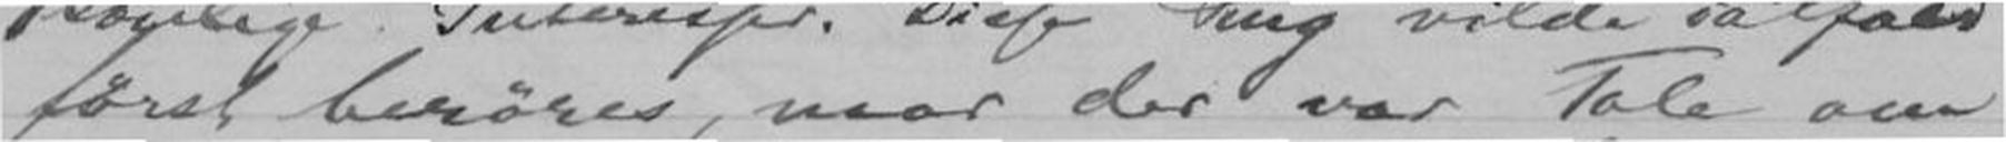først berøres, naar der var Tale om
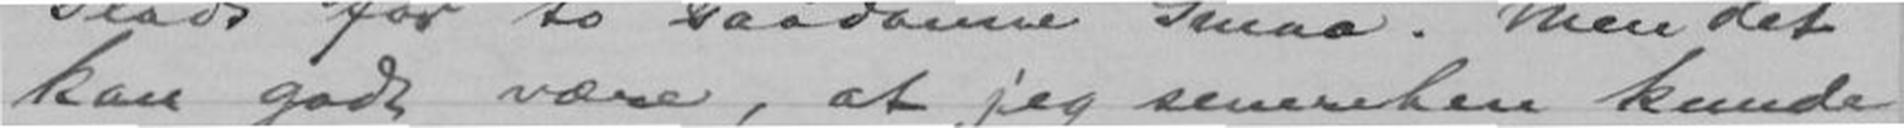kan godt være, at jeg senerehen kunde
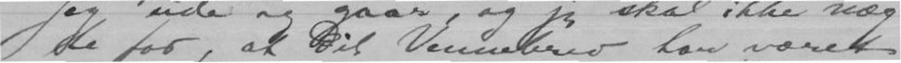te for, at Dit Vennebrev har været
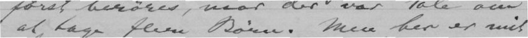at tage flere Børn. Men her er mit
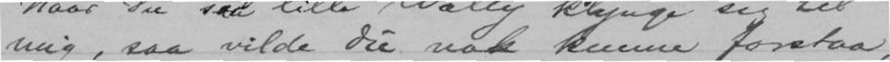mig, saa vilde Du nok kunne forstaa,
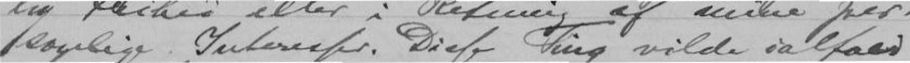sonlige Interesser. Disse Ting vilde ialfald
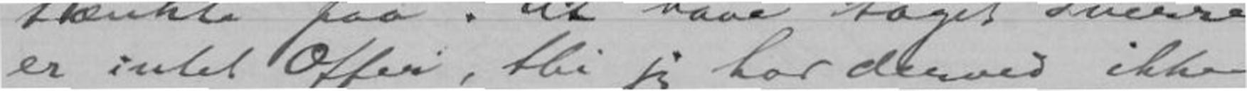er intet Offer, thi jeg har derved ikke
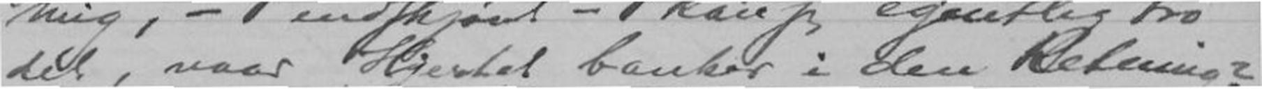det, naar Hjertet banker i den Retning?
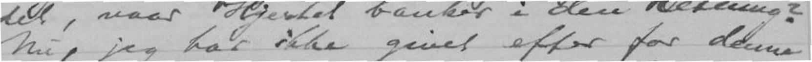Nei, jeg har ikke givet efter for denne
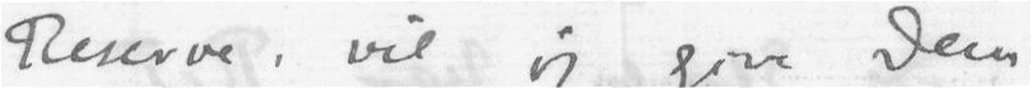Reserve, vil jeg give Dem
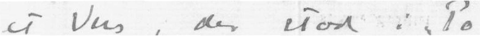et Vers, der stod: Po
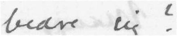bedre sig?
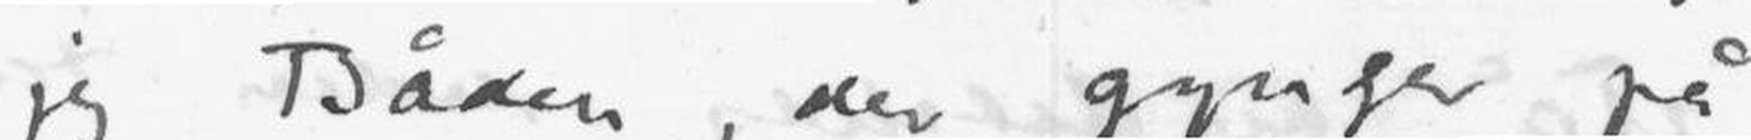jeg Båden, der gynger på
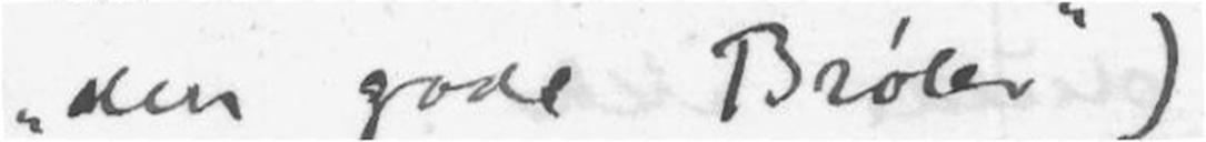den gode Brøler)
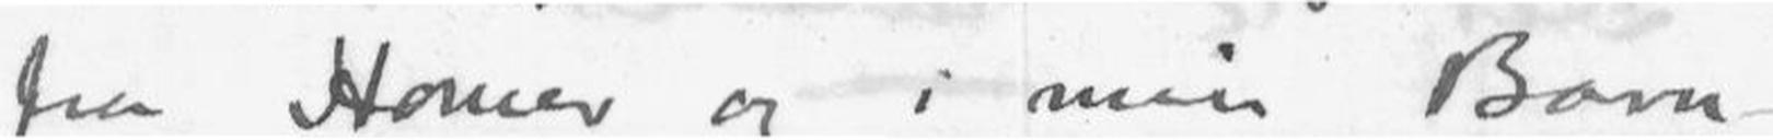fra Homer og i min Barn
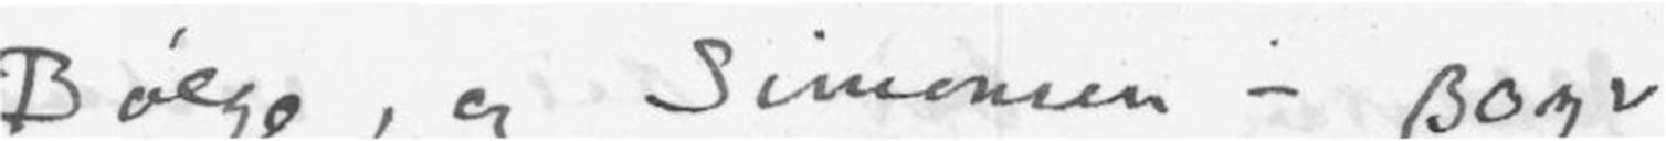Bølge, og Simonsen - Bozr
